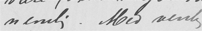nemlig. Med venlig
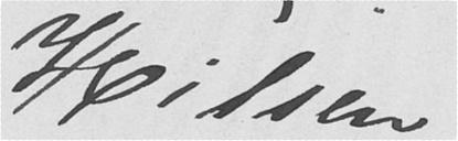Hilsen
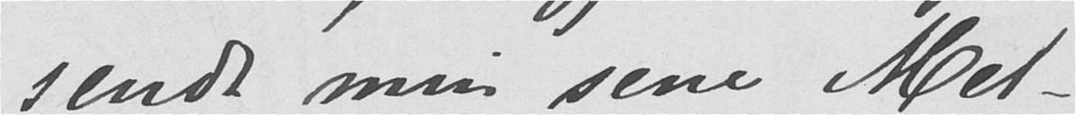sendt min sene Mel-
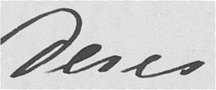Deres
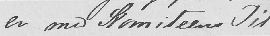er med Komiteens Til-
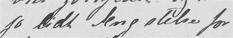jo lidt Ængstelse for
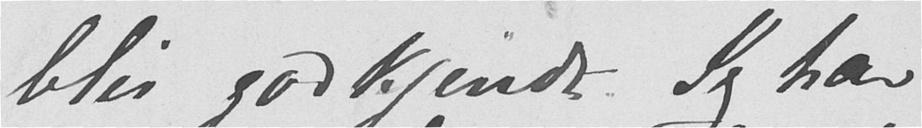blir godkjendt. Jeg har
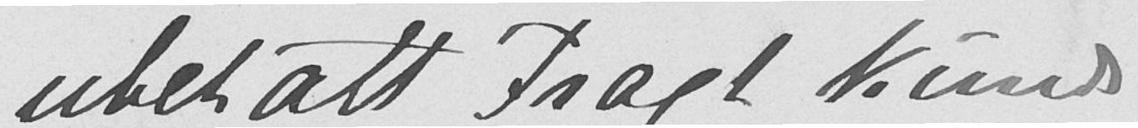ubetalt Fragt kunde
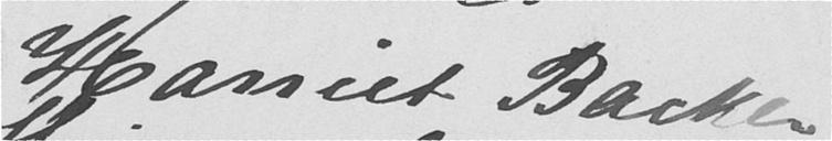Harriet Backer
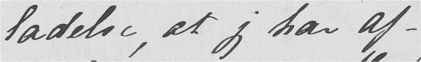ladelse, at jeg har af-
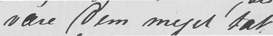være Dem meget tak-
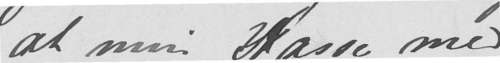at min Kasse med
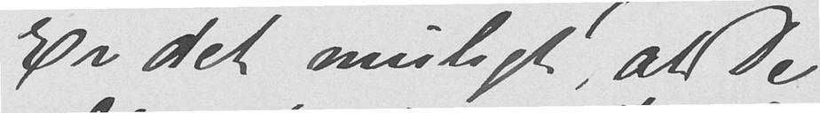Er det muligt, at De
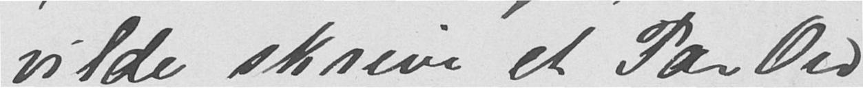vilde skrive et Par Ord
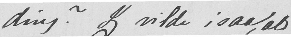ding? Jeg vilde isaafald
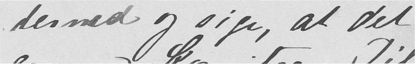derned og sige, at det
